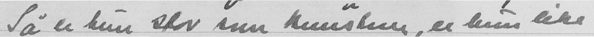Så er hun stor som kunstner, er hun like
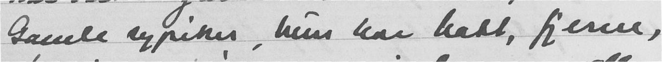Gamle sypiker, hun har hatt, fjerne,
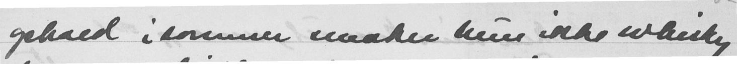ophold isommer smaker hun ikke ikisker
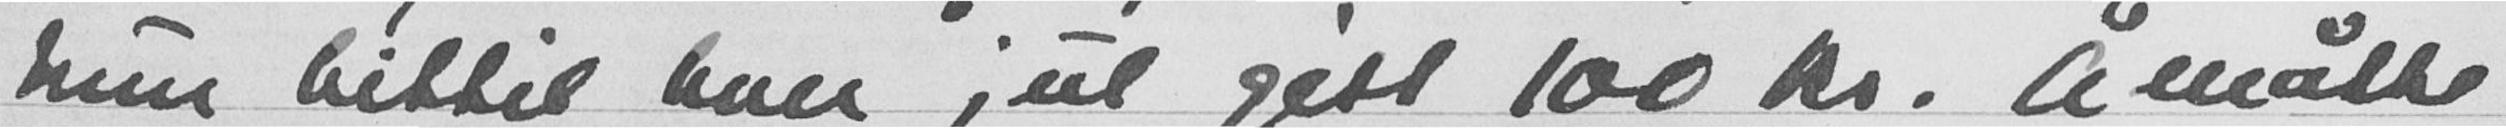hun hittil hver jul gitt 100 kr. Å måtte
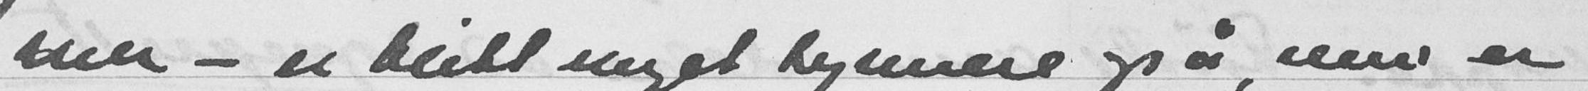mer - er blitt meget tynnere også, men er
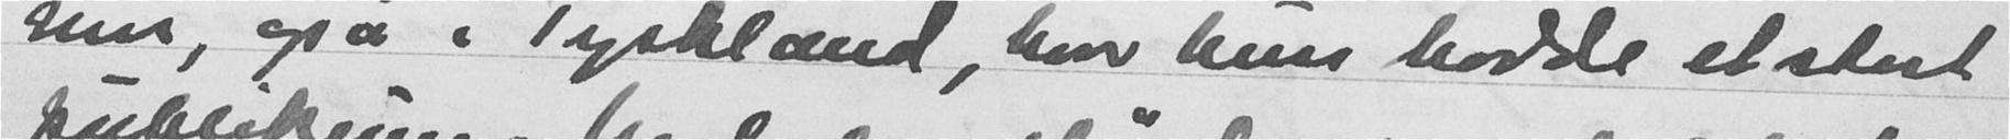inn, også i Tyskland, hvor hun hadde et stort
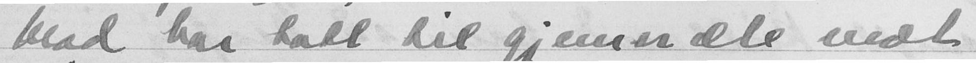blad har talt til gjemnedle mot
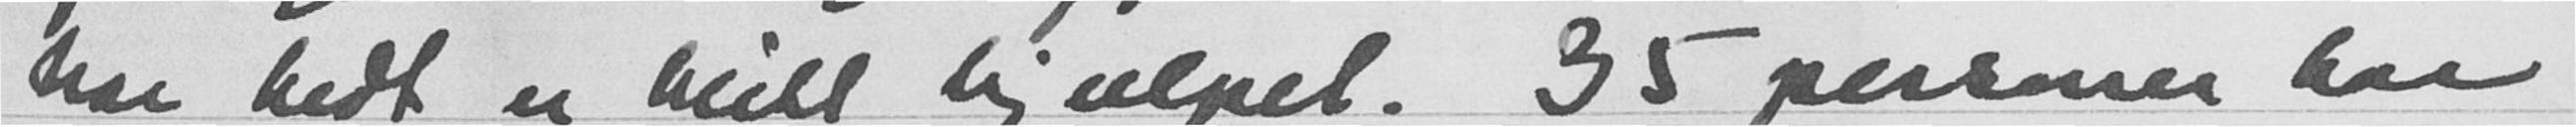har bedt er blitt hjelpet. 35 personer har
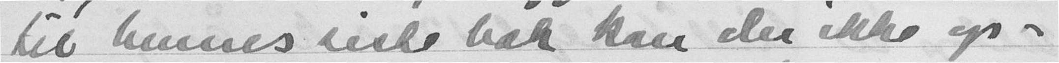til hennes siste bok kan der ikke op-
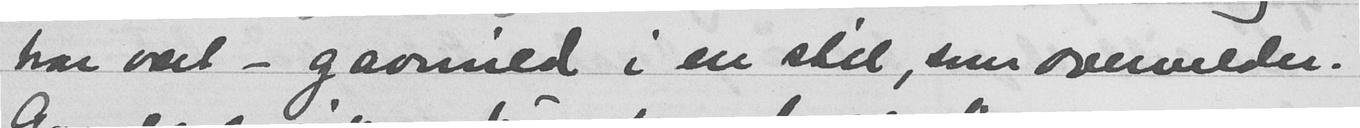har vært - gavmild i en stil, som overvelder.
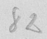88
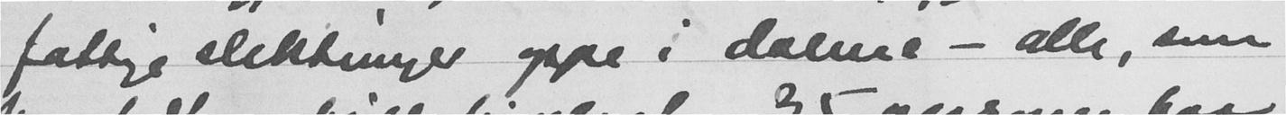fattige slektninger oppe i dalene - alle, som
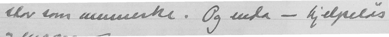stor som menneske. Og enda - hjelpeløs
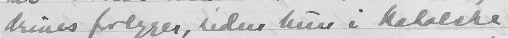drives forlegger, siden hun i katolske
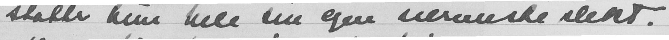støtter hun hele sin armenske slekt.
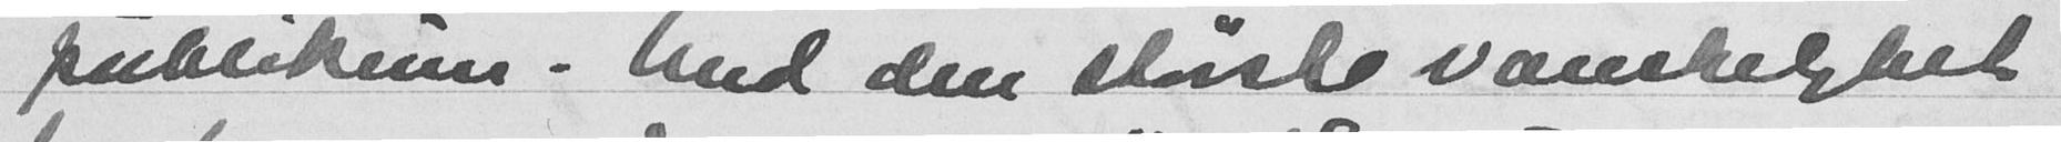publikum. Med den største vanskelighet
